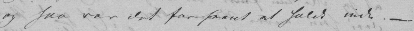og saa var det for seent at holde inde. –
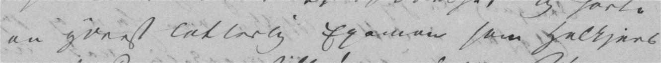en yderst latterlig Examen som Halkjers
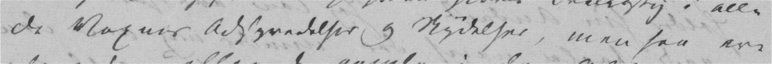de Voxnes Adspredelser og Nydelser, men saa ere
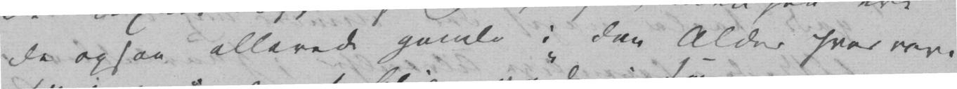de ogsaa allerede gamle i den Alder hvor vore
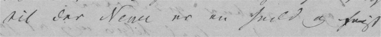til der Neve er en snild og fvist
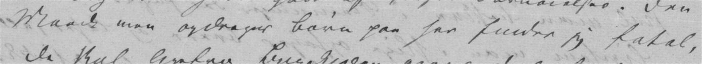Maade man opdrager Børn paa her finder jeg fatal,
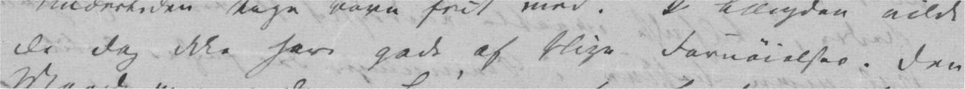de dog ikke have godt af slige Fornøielser. Den
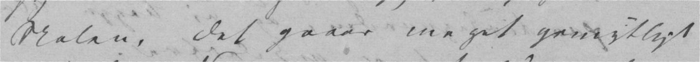Skolen, det gaaer meget gemytligt
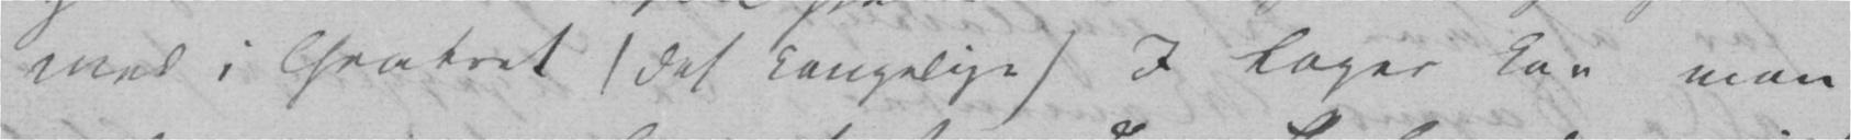med i Theatret (det kongelige) I Loger kan man
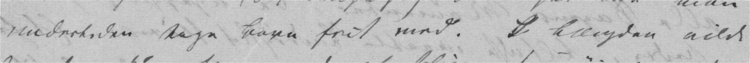undertiden tage Børn frit med. I Længden vilde
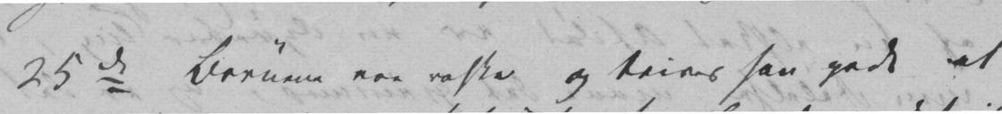25de Børnene ere raske og trives saa godt at
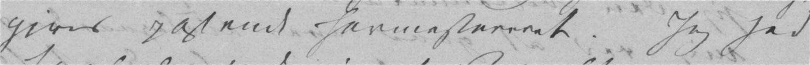gives passende hovmestereret. Jeg sad
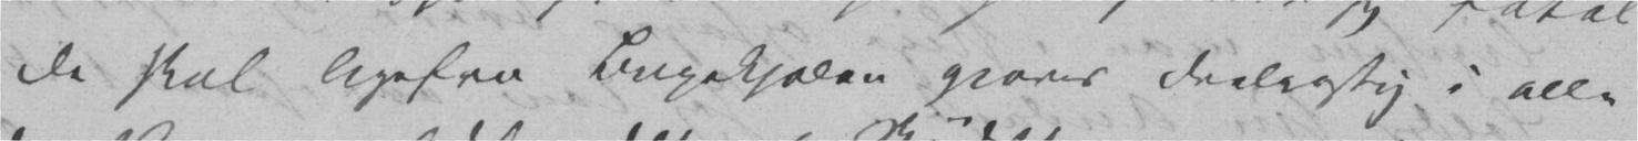de skal ligefra Buxekjolen gjøres deelagtig i alle
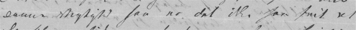denne Vigtighed saa er det ikke saa frit at
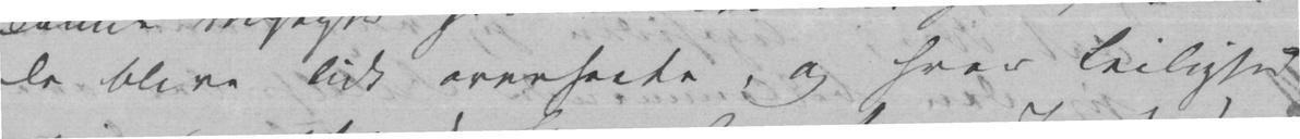de blive lidt overseete, og hvor Leilighed
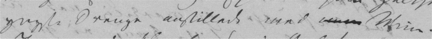yngste Drenge anstillede med mine Mine.
